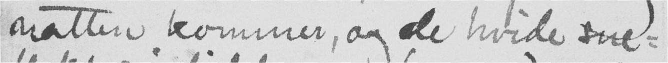natten kommer, og de hvide sne-
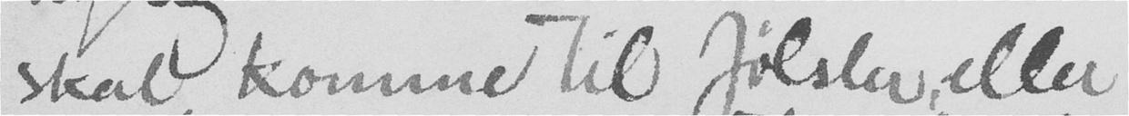skal komme til Jølster eller
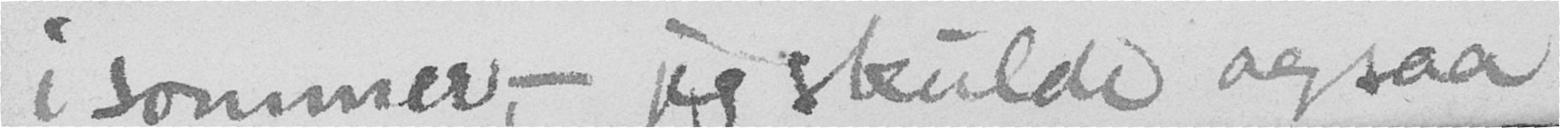i sommer,- jeg skulde ogsaa
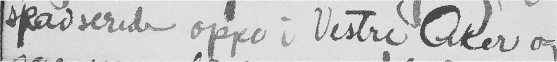spadserede oppe i Vestre Aker og
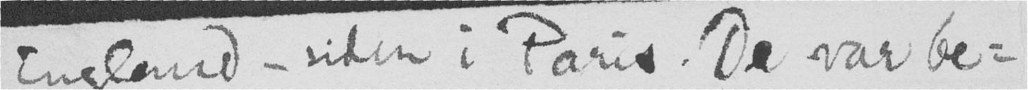England - siden i Paris. De var be-
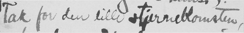Tak for den lille stjerneblomsten,
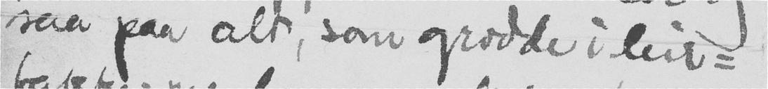saa paa alt, som grodde i leir-
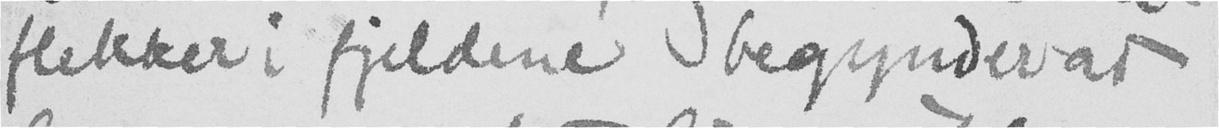flekker i fjeldene begynder at
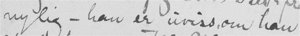nylig, - han er uviss, om han
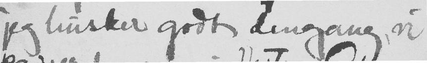jeg husker godt dengang, vi
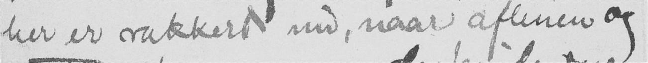her er vakkert nu, naar aftenen og
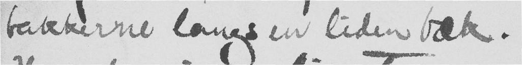bakkerne langs en liden bæk.
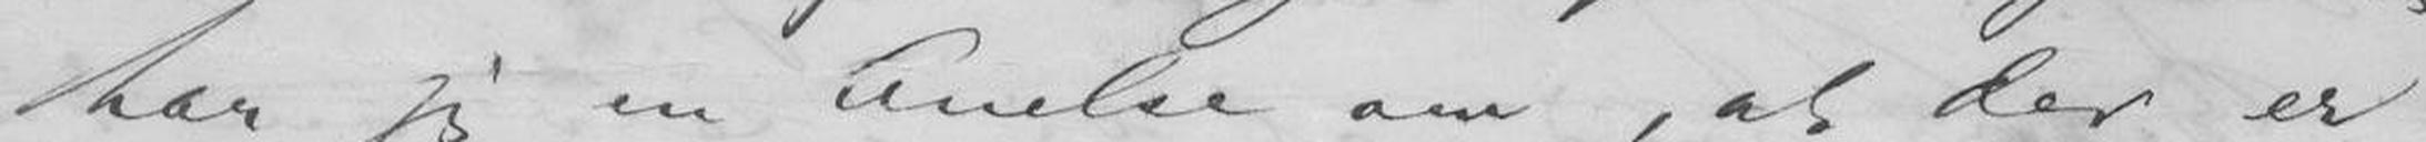har jeg en Anelse om, at der er
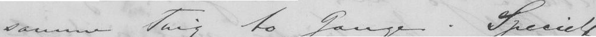samme Ting to Gange. Specielt
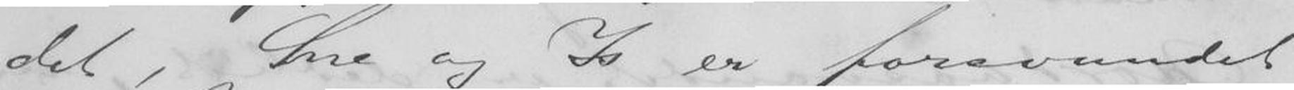det, Sne og Is er forsvundet
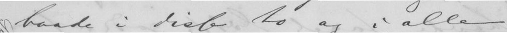baade i disse to og i alle
